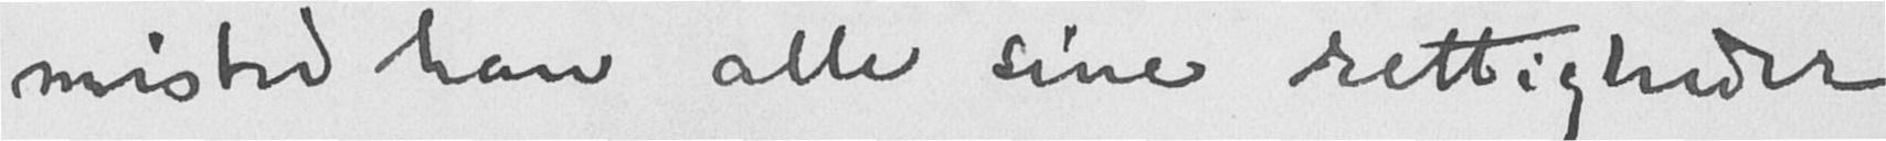misted han alle sine rettigheder
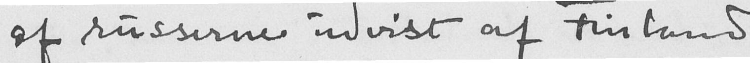af russerne udvist af Finland,
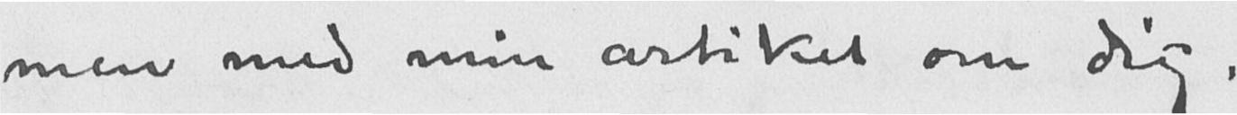men med min artikel om dig.
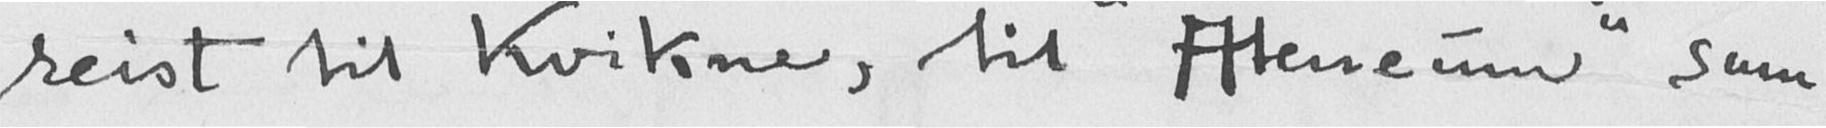reist til Kvikne, til "Ateneum" sam-
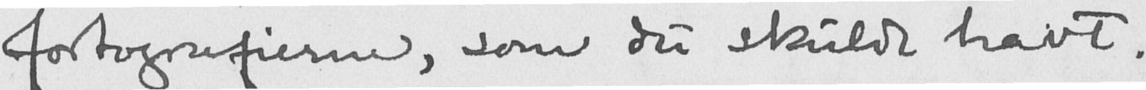fortografierne, som du skulde havt.
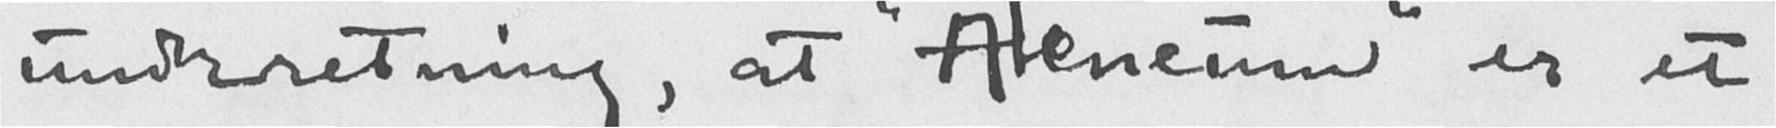underretning, at "Ateneum" er et
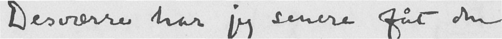Desværre har jeg senere fåt den
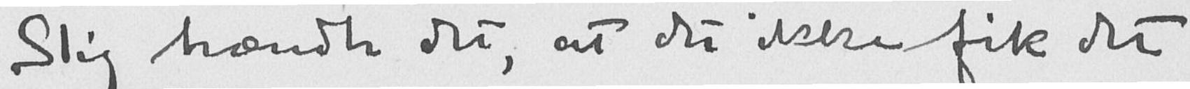Slig hændte det, at du ikke fik det
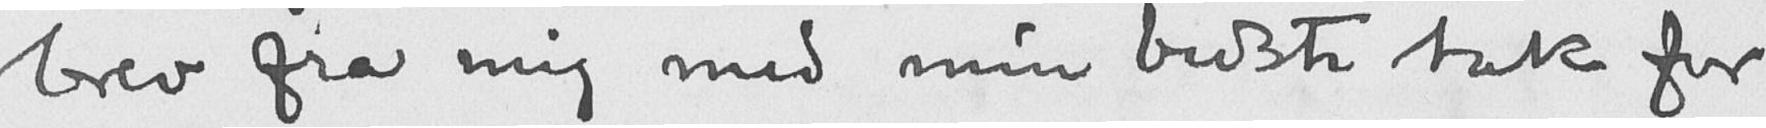brev fra mig med min bedste tak for
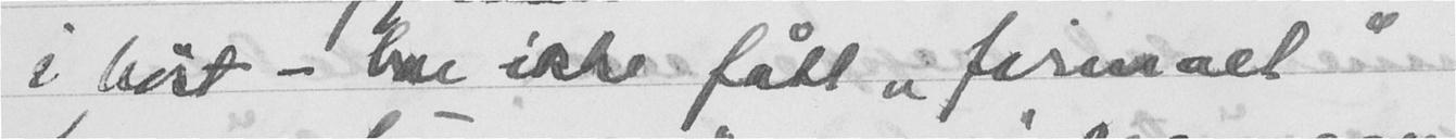i høst - har ikke fått "firmaet"
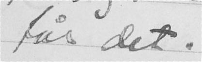får det.
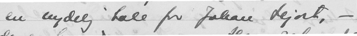en nydelig tale for Johan Hjort, -
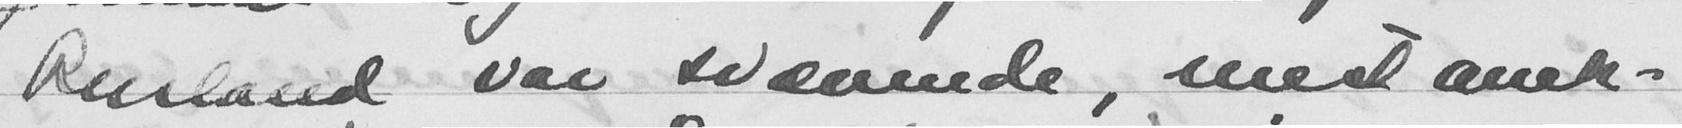Rusland var svævende, med anek-
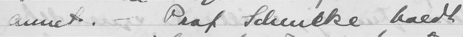annet. - Prof Schulke holdt
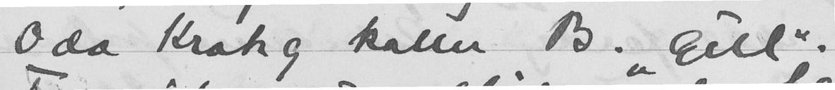Oda Krohg kaller B. "gul".
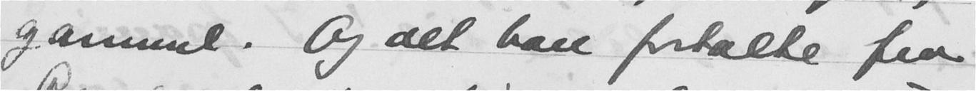gammel. Og alt han fortalte fra
